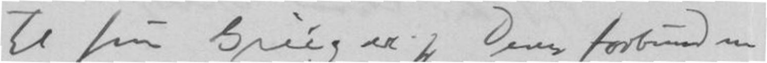til fru Grieg er jeg Deres forbundne
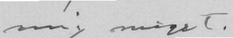mig meget.
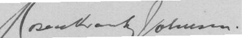Rosenkrantz Johnsen.
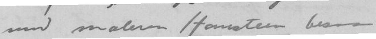med maleren Hamsteen besaa
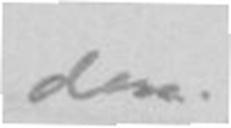den.
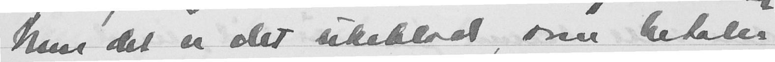Men det er det ukeblad, som betaler
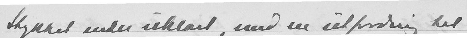Stykket ender uklart, med en utfordring til
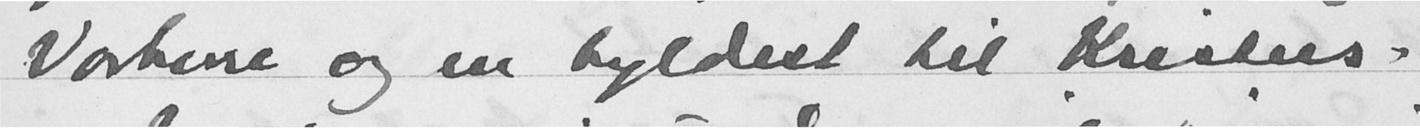Vorherre og en hyldest til Kristus.
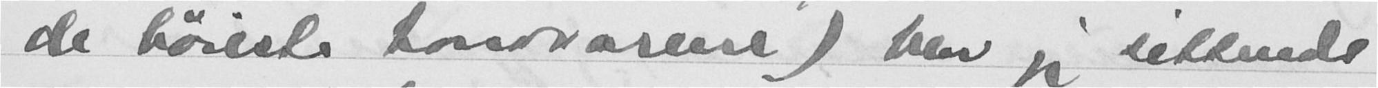de høieste honorarene) blev jeg sittende
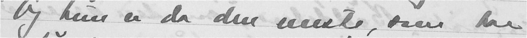Og hun er da den eneste, som har
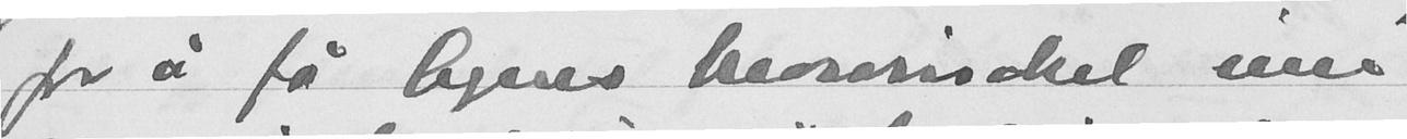for å få Agnes Mowinckel inn
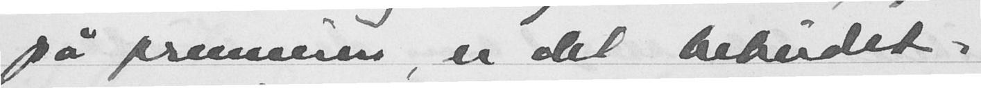på premieren, er det bebudet.
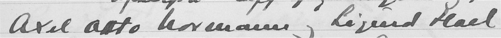Axel Otto Normann og Sigurd Hoel
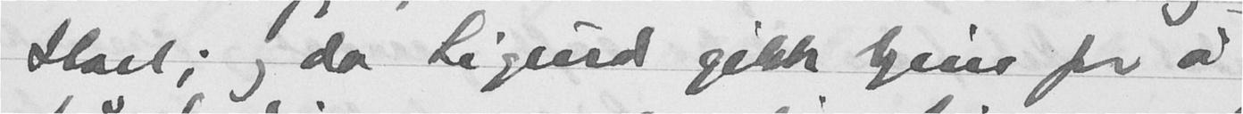Hoel; og da Sigurd gikk hjem for å
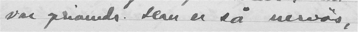var oprivende. Han er så nervøs,
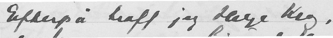Efterpå traff jag Helge Krog,
